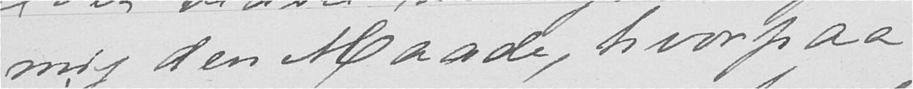mig den Maade, hvorpaa
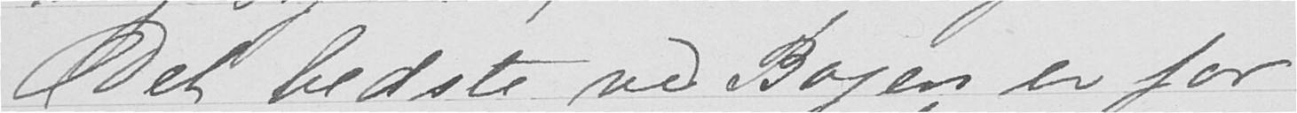Det bedste ved Bogen er for
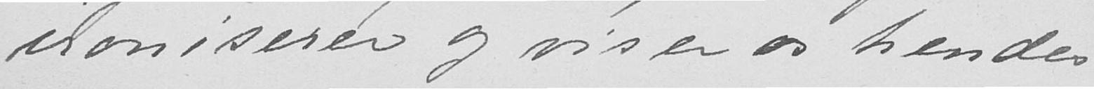ironserer og viser os hendes
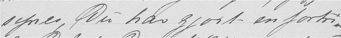synes Du har gjort en fortrin-
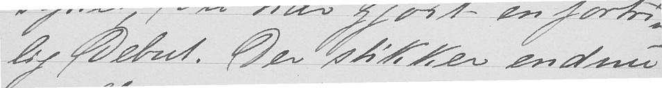lig Debut. Der stikker endnu
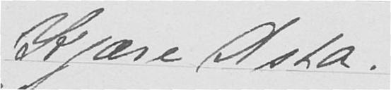Kjære Asta.
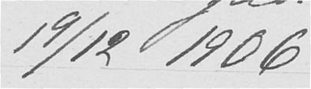19/12 1906.
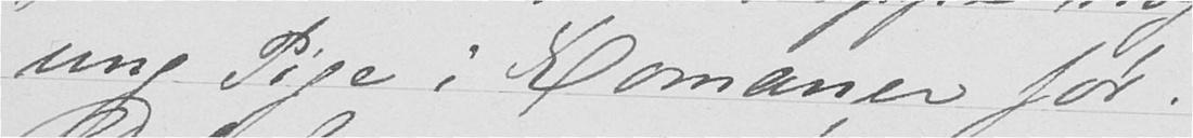ung Pige i Romaner før.
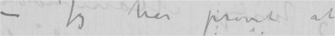– Jeg har prøvet at
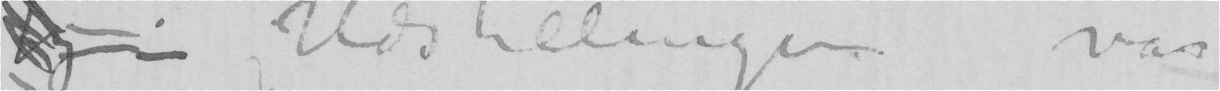Jeg Udstillingen var
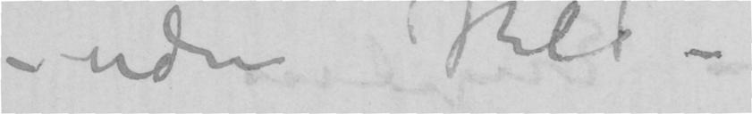uden Held –
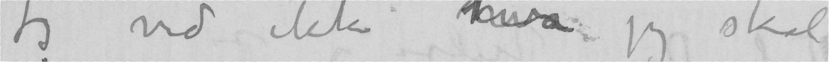Jeg ved ikke hva jeg skal
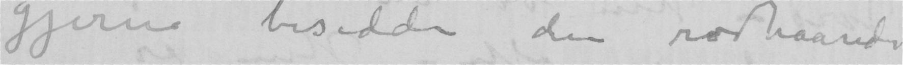gjerne besidde den rødhaarede
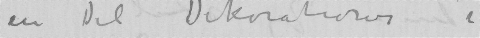en Del Dekorationer i
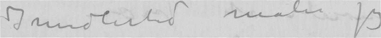Imidlertid maler jeg
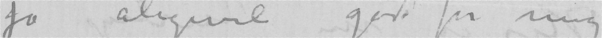jo aligevel godt for mig
